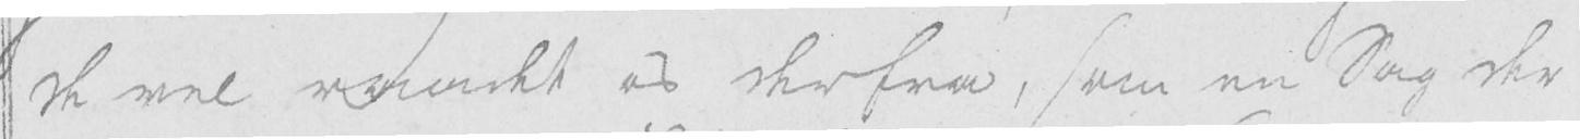de vel vendet os derfra, som en Sag der
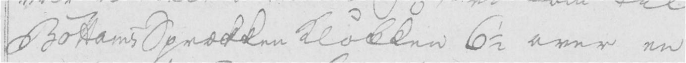Bottams Sprækken Klokken 6 1/2 over en
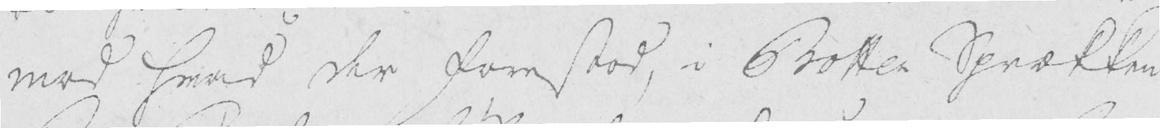mod hvad der forestod, i Botter Sprækken
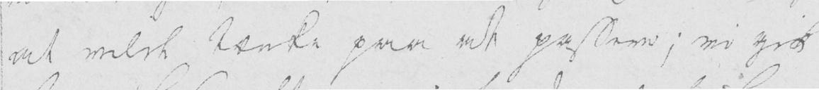at vilde tænke paa at passere; vi gik
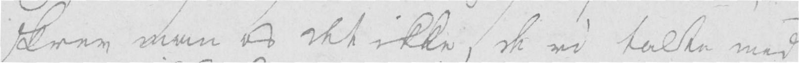skrev man os det ikke, de vi talte med
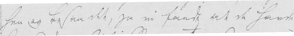hen og besaa det, ja vi fandt at de havde
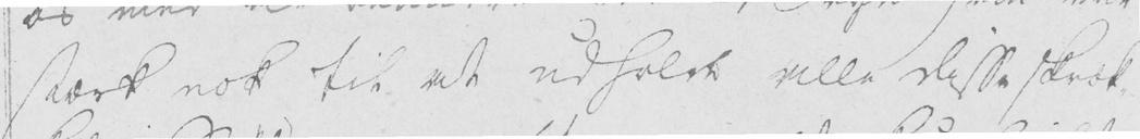stærk nok til at udholde alle disse skræk-
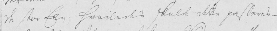de stor Elv; hvorledes skulde dette passeres -
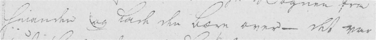hinanden og lade den bære over - det var
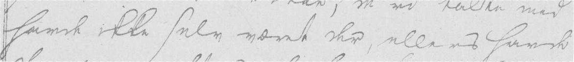havde ikke selv været der, ellers havde
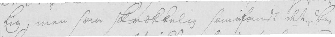lig, men saa skrækkelig som vi fandt det, be-
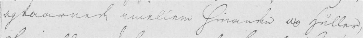og taarnede imellem hinanden og Huller,
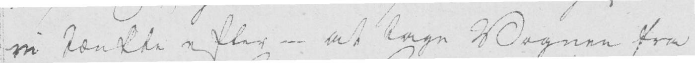vi tænkte efter - at tage Vognen fra
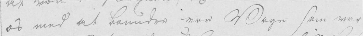os med at beundre vor Vogn som var
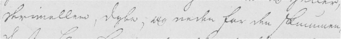derimellem, dybe, og neden for den skummen-
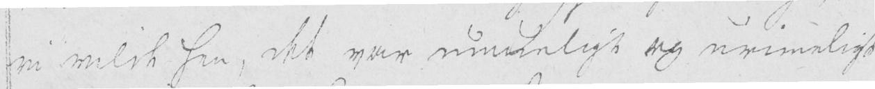vi vilde hen, det var umueligt og urimeligt
